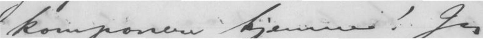komponere hjemme! Jeg
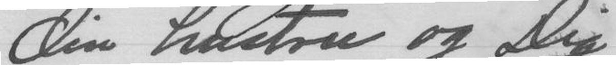din hustru og Dig
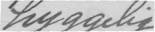hyggelig
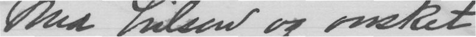Med hilsen og ønsket
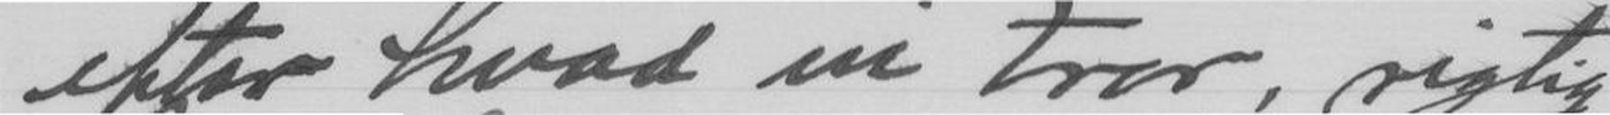efter hvad vi tror, rigtig
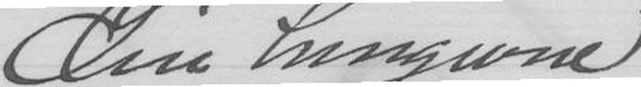din hengivne
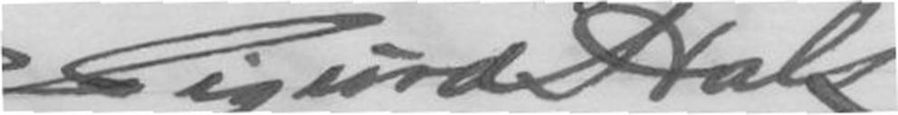Sigurd Hals
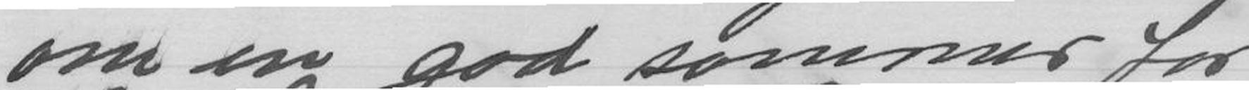om en god sommer for
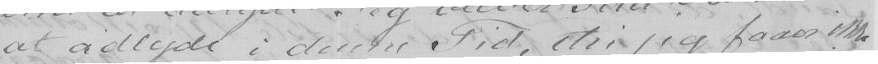at adlyde i denne Tid, thi jeg faaer ikke
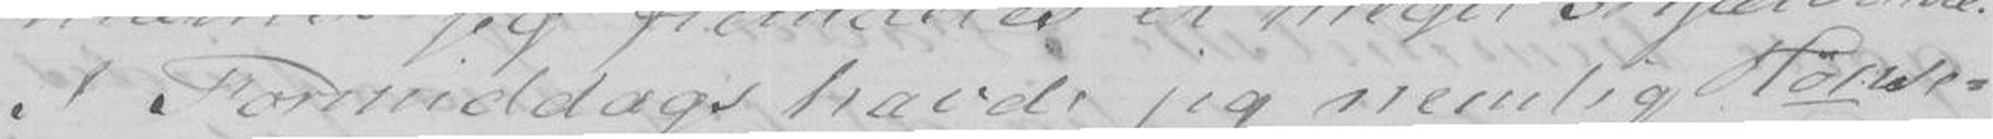I Formiddags havde jeg nemlig Hørse-
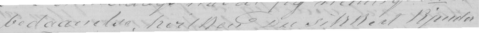bedaanelse, hvilket Du sikkert kjender
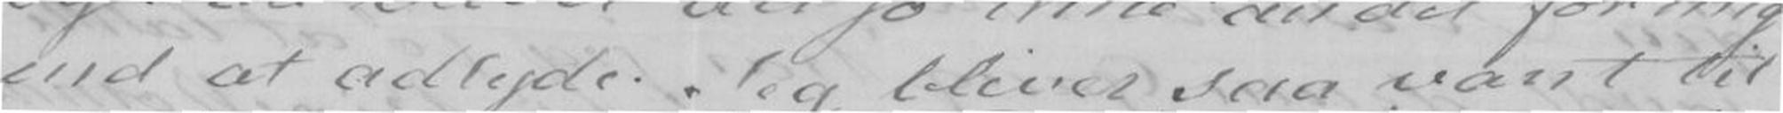end at adlyde. Jeg bliver saa vant til
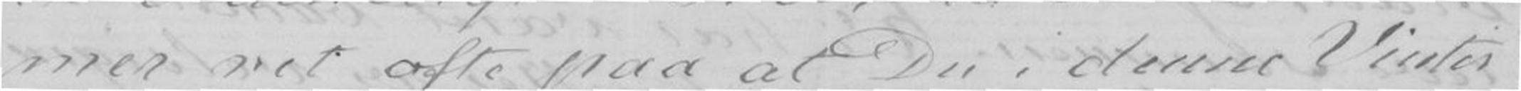mer ret ofte paa at Du i denne Vinter
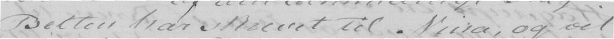Betten har skrevet til Nina, og vil
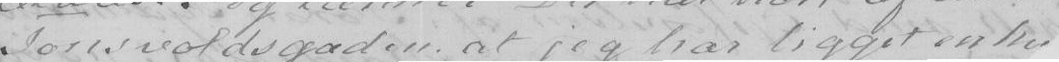Jonsvoldsgaden at jeg har ligget en he
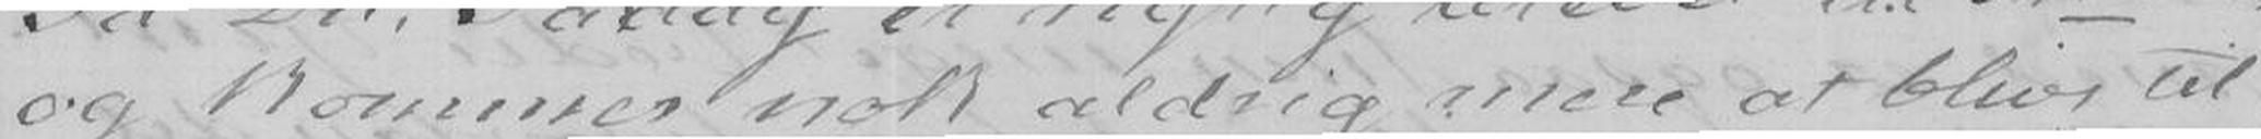og kommer nok aldrig mere at blive til
